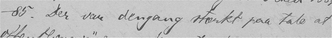-85. Der var dengang stærkt paa tale at
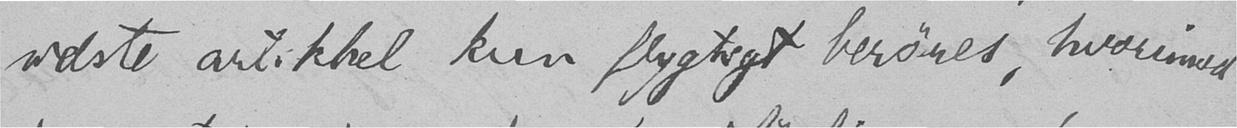sidste artikkel kun flygtigt berøres, hvorimod
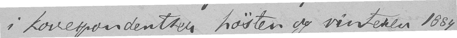i korrespondentser høsten og vinteren 1884
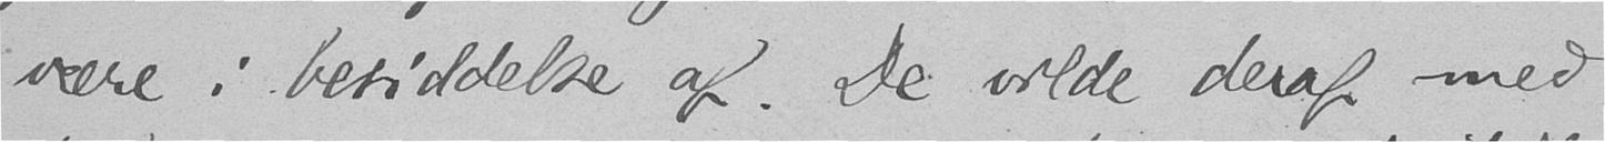være i besiddelse af. De vilde deraf med
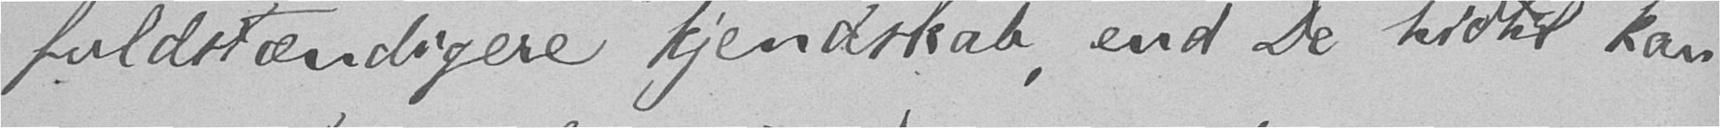fuldstændigere kjendskab, end De hidtil kan
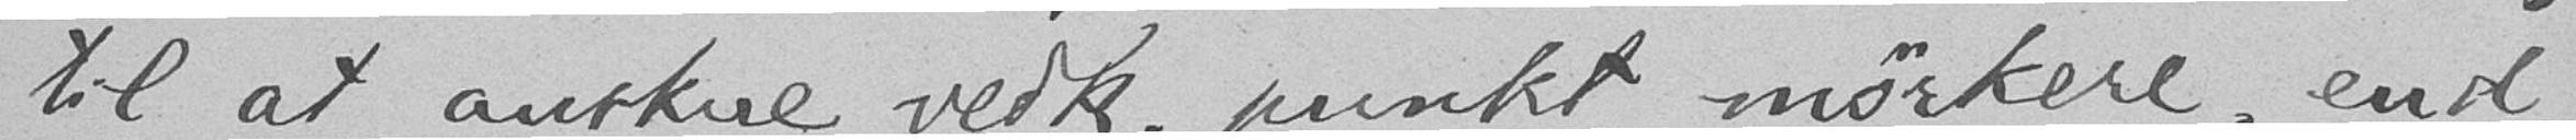til at anskue vedk. punkt mørkere, end
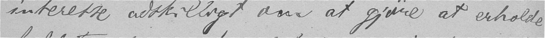interesse adskilligt om at gjøre at erholde
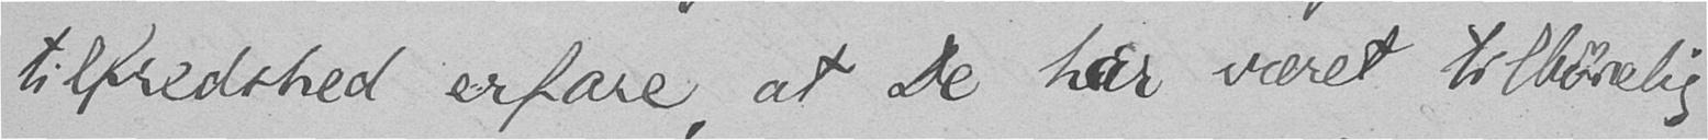tilfredshed erfare, at De har været tilbøielig
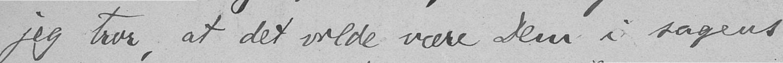jeg tror, at det vilde være Dem i sagens
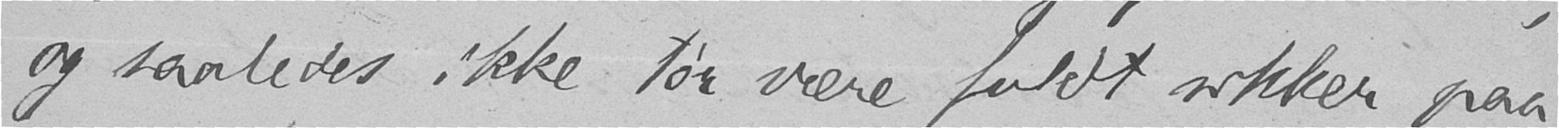og saaledes ikke tør være fuldt sikker paa,
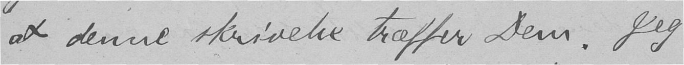at denne skrivelse træffer Dem. Jeg
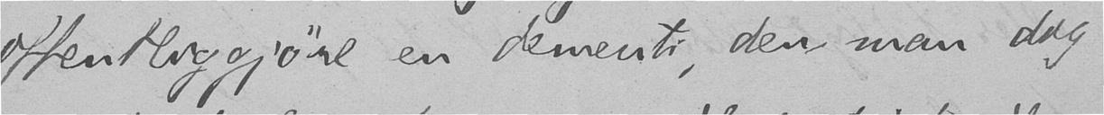offentliggjøre en dementi, den man dog
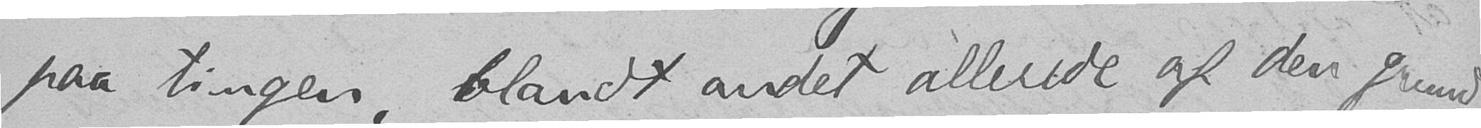paa tingen, blandt andet allerede af den grund,
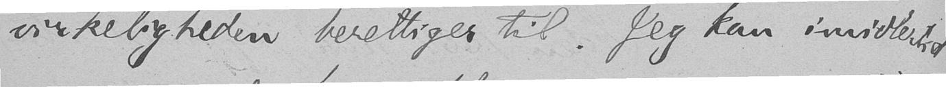virkeligheden berettiger til. Jeg kan imidlertid
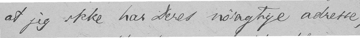at jeg ikke har Deres nøiagtige adresse,
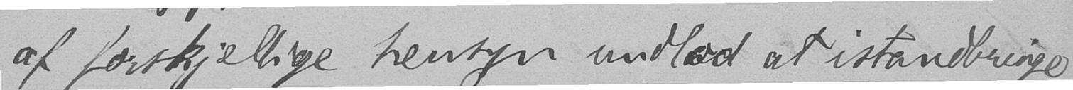af forskjellige hensyn undlod at istandbringe.
